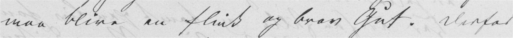maa blive en flink og brav Gut. Derfor
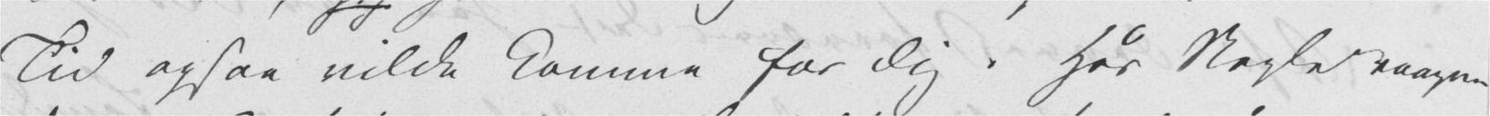Tid ogsaa vilde komme for Dig. Hos Nogle vaagner
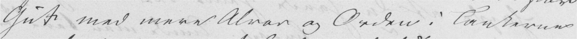Gut med mere Alvor og Orden i Tankerne
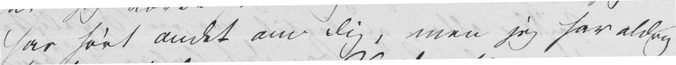har hørt andet om Dig, men jeg har aldrig
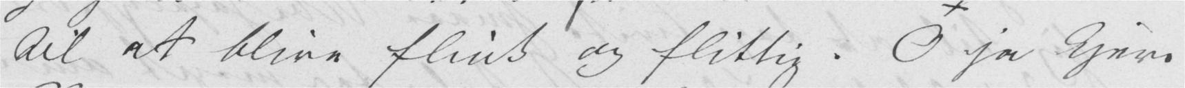til at blive flink og flittig. O ja kjere
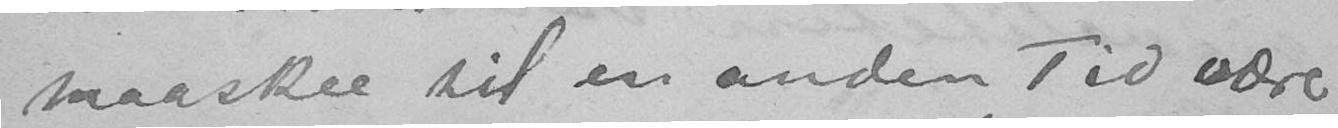maaskee til en anden Tid være
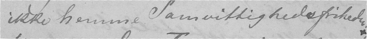ikke hemme Samvittighedsfriheden.
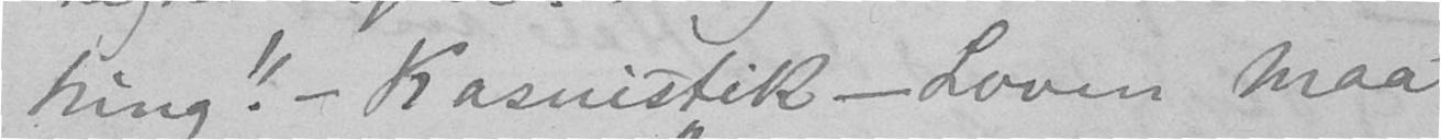ning." - Kasuistik - Loven maa
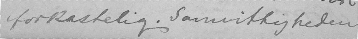forkastelig. Samvittigheden
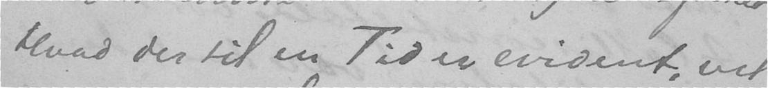Hvad der til en Tid er evident, vil
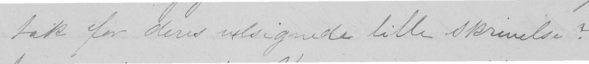tak for deres velsignede lille skrivelse?
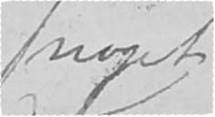noget
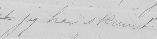jeg har skrevet
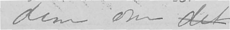dem om det
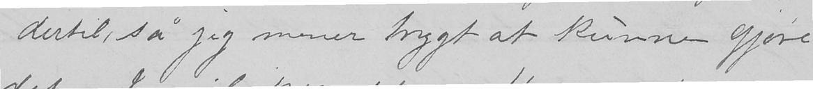dertil, så jeg mener trygt at kunne gjøre
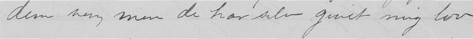dem ven, men de har selv givet mig lov
